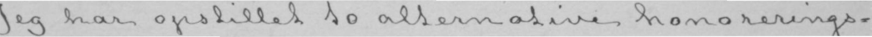Jeg har opstillet to alternative honorerings-
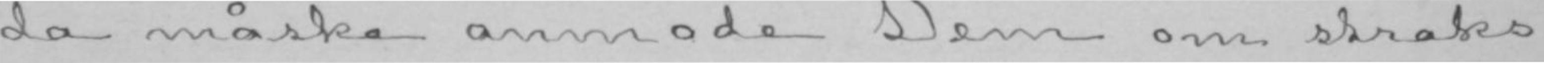da måske anmode Dem om straks
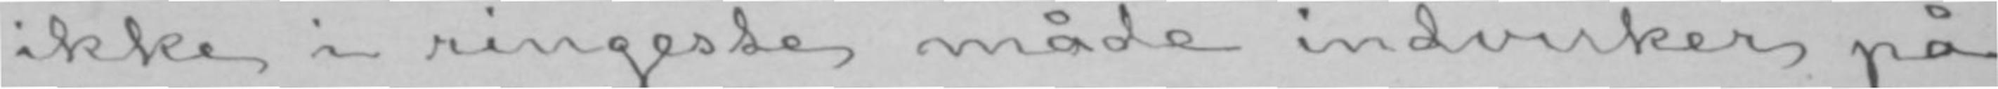ikke i ringeste måde indvirker på
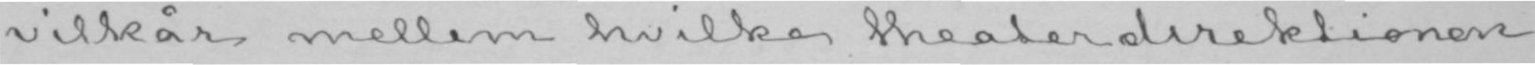vilkår mellem hvilke theaterdirektionen
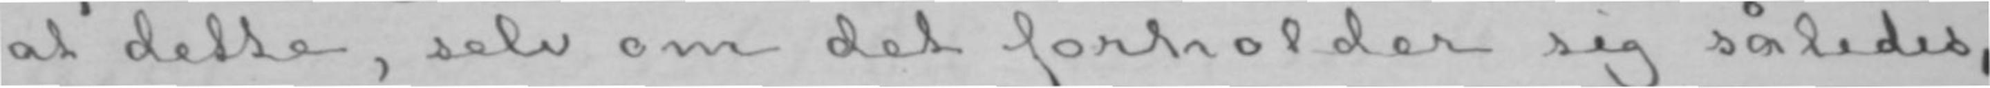at dette, selv om det forholder sig således,
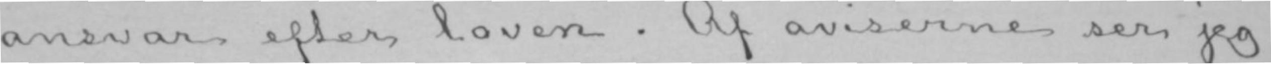ansvar efter loven. Af aviserne ser jeg
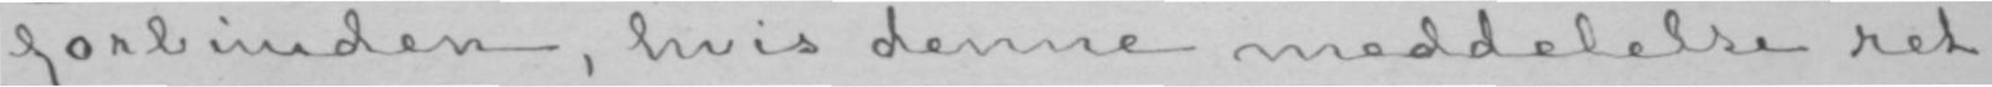forbunden, hvis denne meddelelse ret
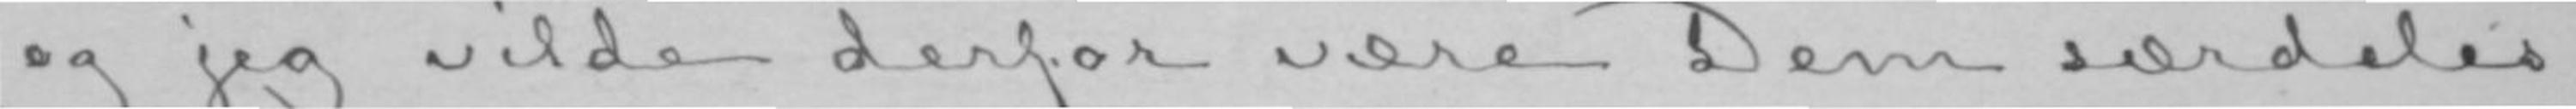og jeg vilde derfor være Dem særdeles
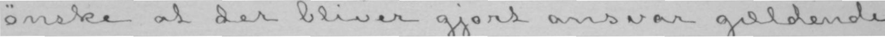ønske at der bliver gjort ansvar gældende
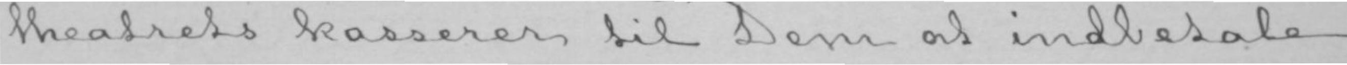theatrets kasserer til Dem at indbetale
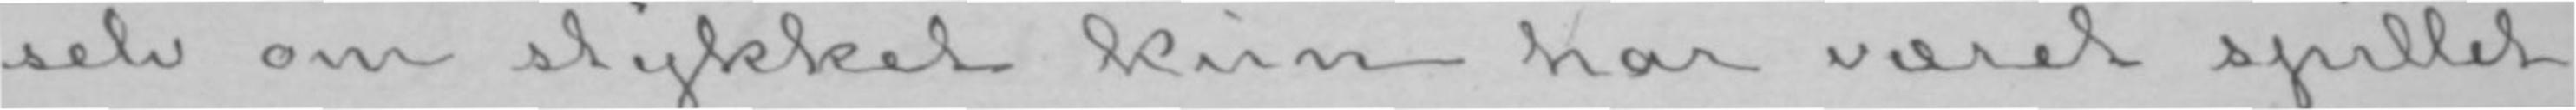selv om stykket kun har været spillet
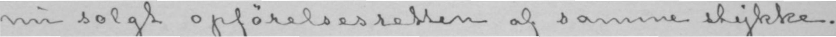nu solgt opførelsesretten af samme stykke.
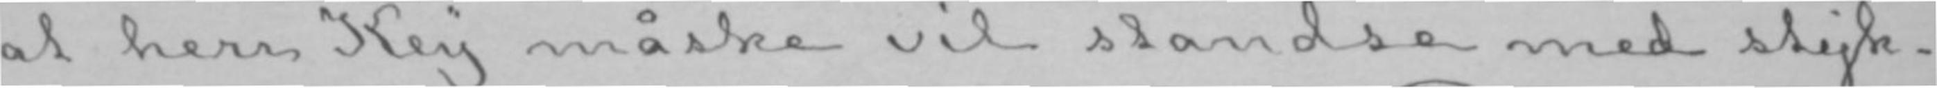at herr Key måske vil standse med styk-
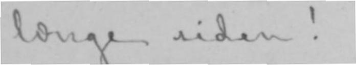længe siden!
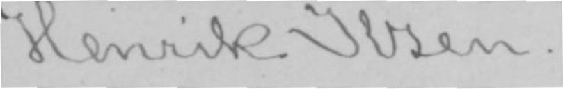Henrik Ibsen.
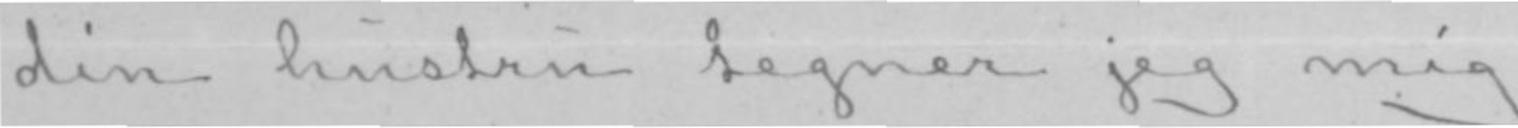din hustru tegner jeg mig
